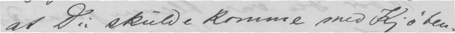at Du skulde komme med Kjøben-
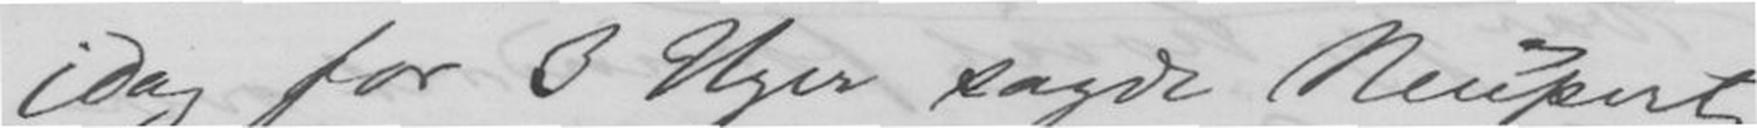idag for 3 Uger sagde Neupert
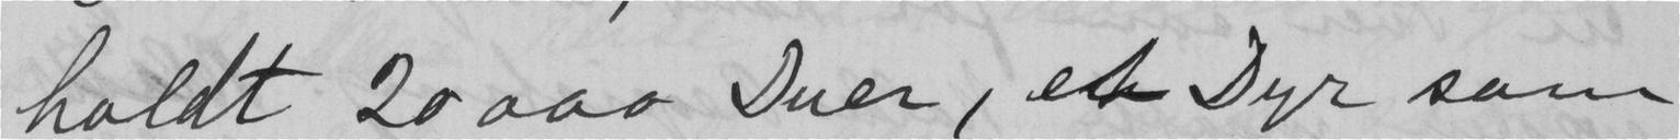holdt 20 000 Duer, et Dyr som
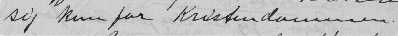sig kun for Kristendommen.
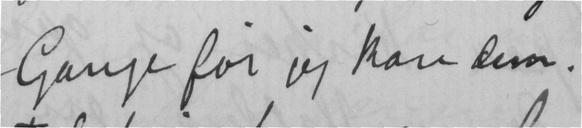Gange før jeg kan dem.
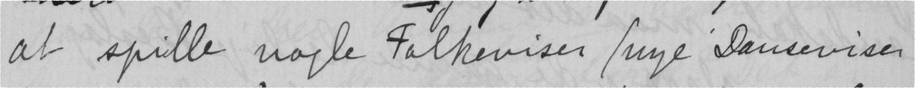at spille nogle Folkeviser (nye Danseviser
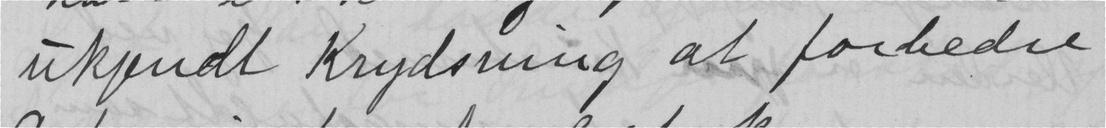ukjendt Krydsning at forbedre
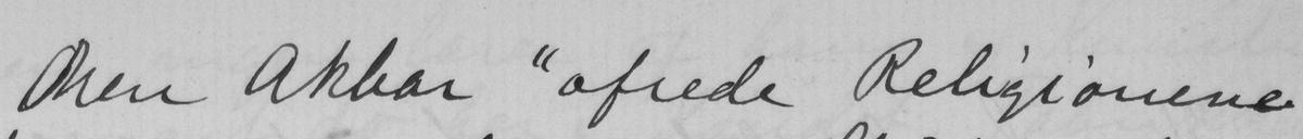Men Akbar "ofrede Religionene"
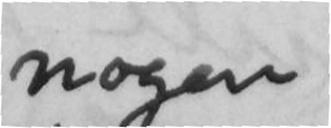nogen
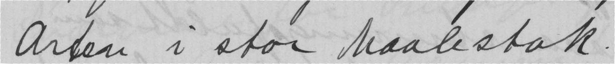Arten i stor Maalestok.
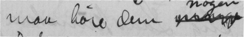maa høre dem
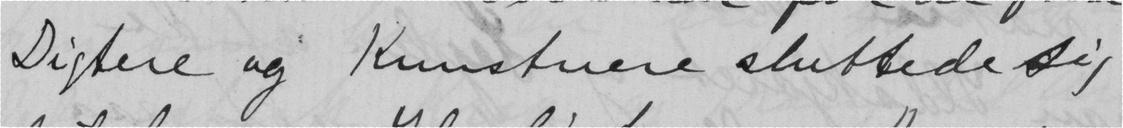Digtere og Kunstnere sluttede sig
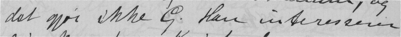det gjør ikke G. Han interesserer
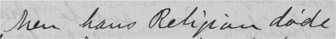Men hans Religion døde
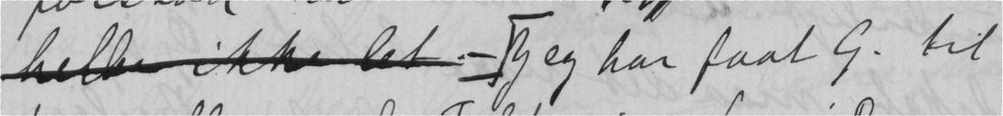Jeg har faat G. til
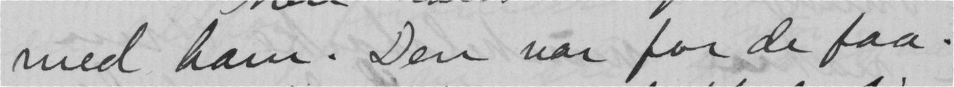med ham. Den var for de faa.
